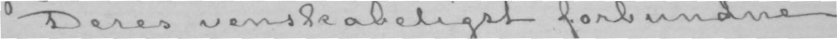Deres venskabeligst forbundne
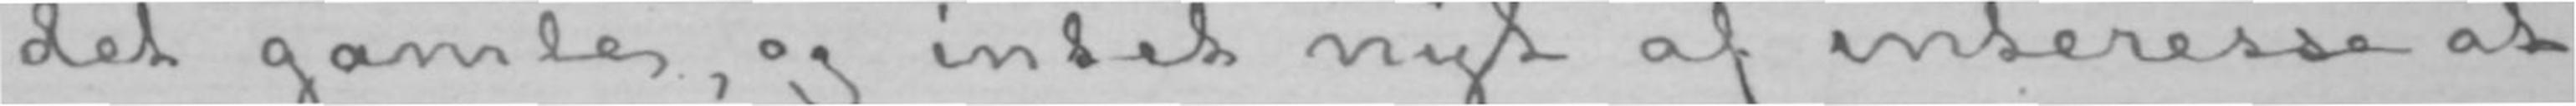det gamle, og intet nyt af interesse at
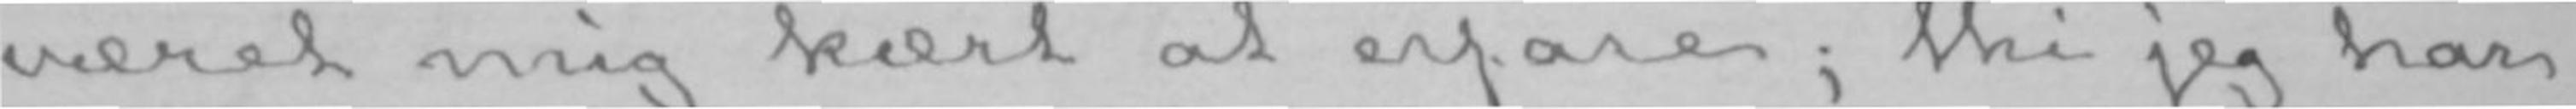været mig kært at erfare; thi jeg har
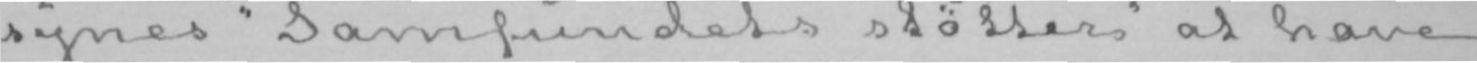synes «Samfundets støtter» at have
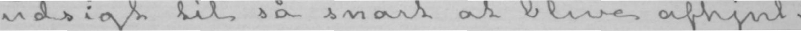udsigt til så snart at blive afhjul-
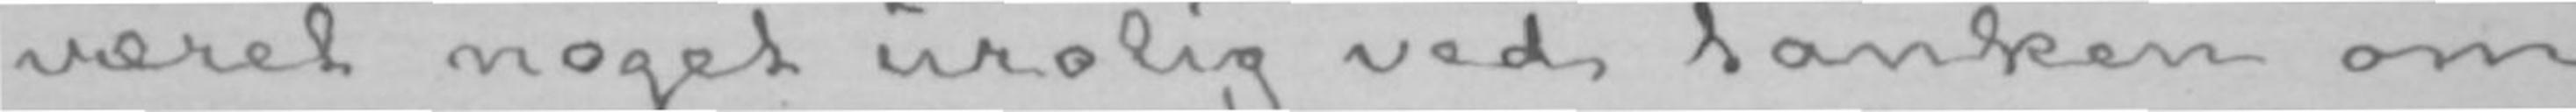været noget urolig ved tanken om
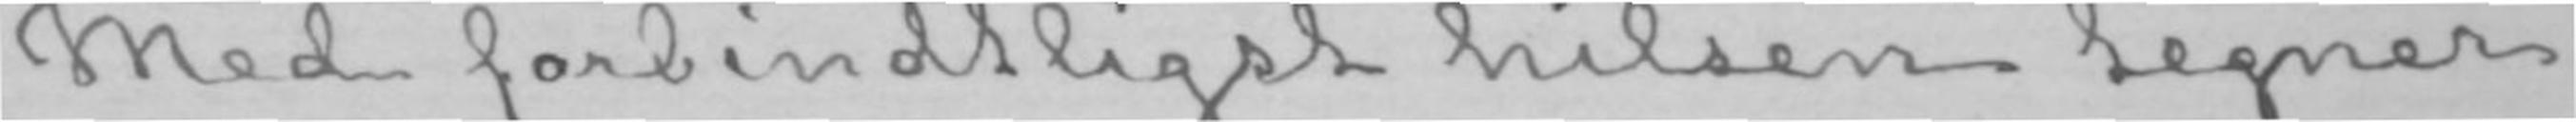Med forbindtligst hilsen tegner
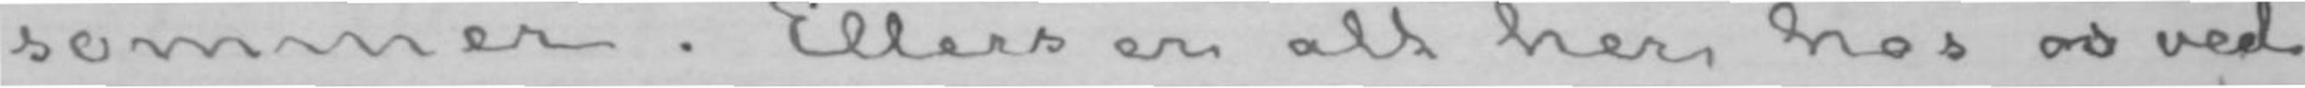sommer. Ellers er alt her hos os ved
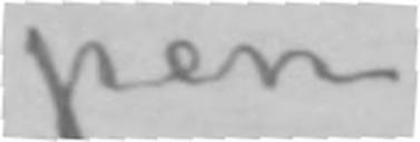pen.
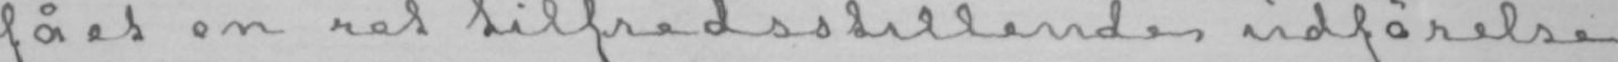fået en ret tilfredsstillende udførelse
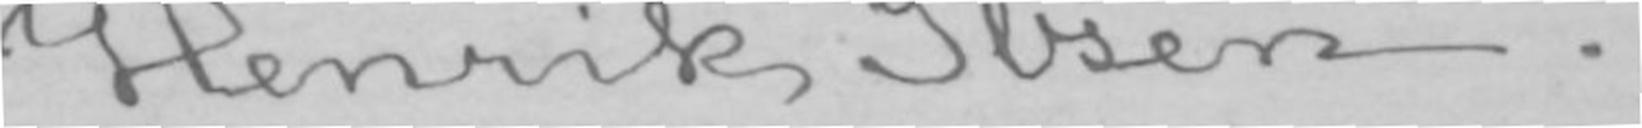Henrik Ibsen.
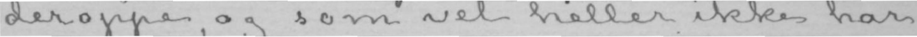deroppe, og som vel heller ikke har
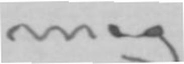jeg mig
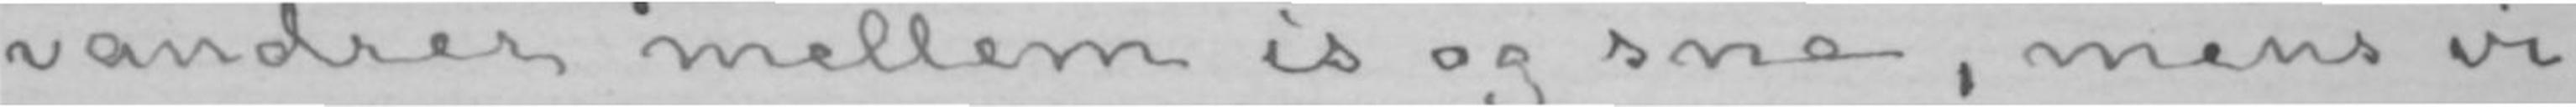vandrer mellem is og sne, mens vi
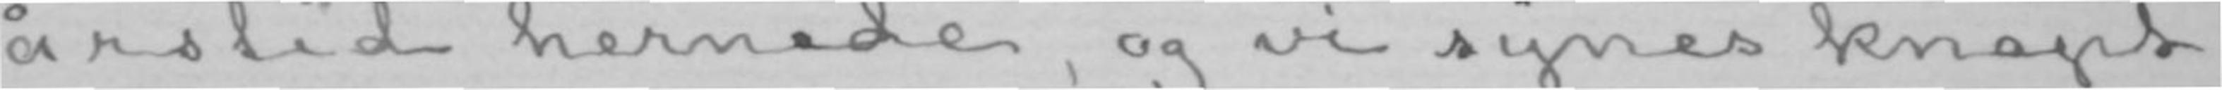årstid hernede, og vi synes knapt
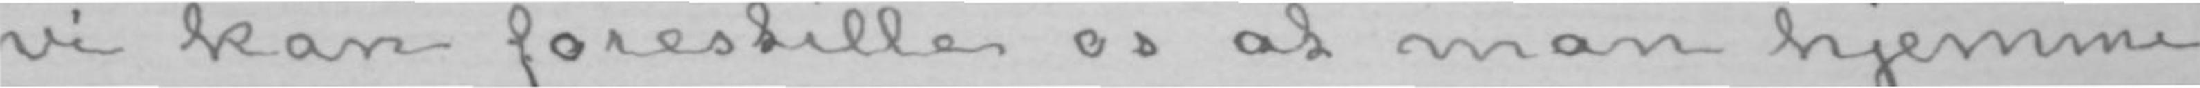vi kan forestille os at man hjemme
